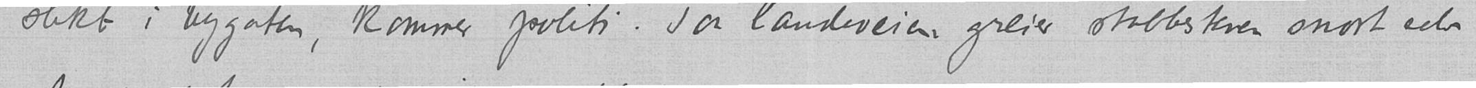slikt i bygaten, kommer politi. Paa landeveien greier stabbestenen snart selv
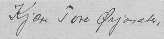Kjære Tore Ørjasæter,
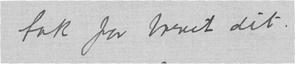tak for brevet dit.
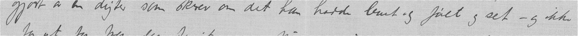gjort av en digter som skrev om det han hadde levet og følt og set – og ikke
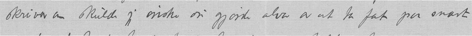skriver om skulde jeg ønske du gjorde alvor av at ta fat paa snart
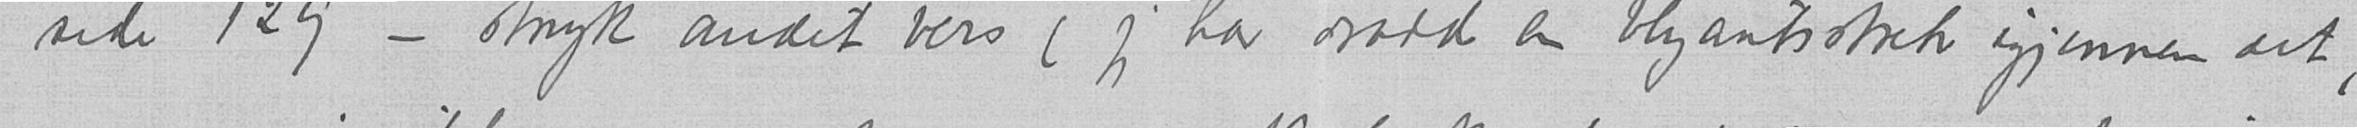side 129 – stryk andet vers (jeg har dradd en blyantsstrek igjennem det,
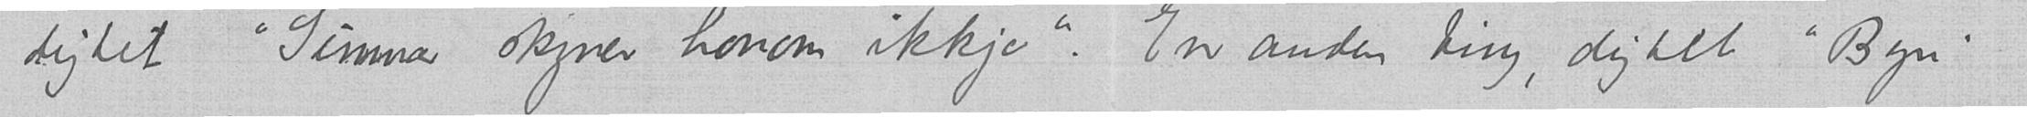digtet "Gunnar skyner honom ikkje". En anden ting, dighet "Byn"
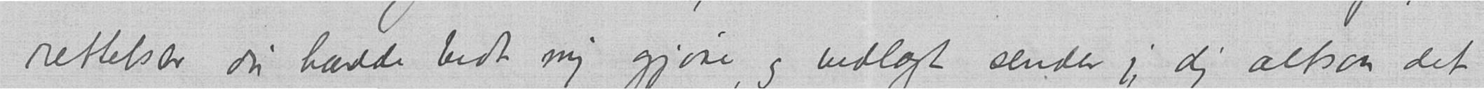rettelser du hadde bedt mig gjøre, og vedlagt sender jeg dig altsaa det
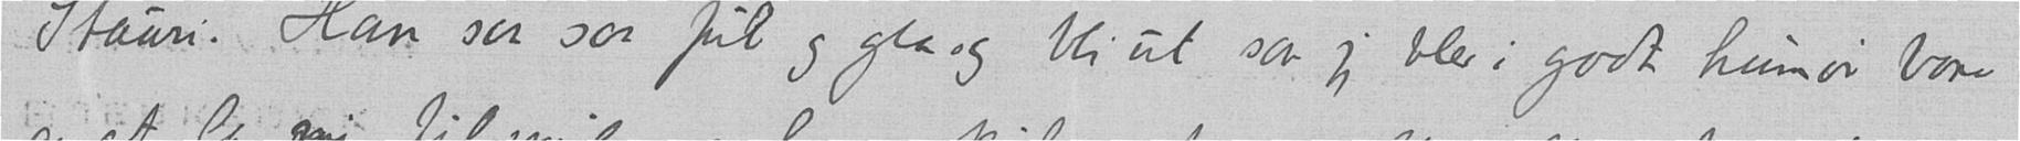Stauri. Han saa saa ful og gla og bli ut, saa jeg blev i godt humør bare
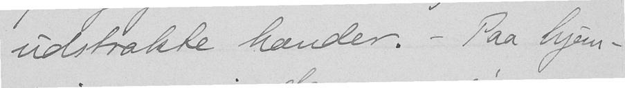udstrakte hænder. - Paa hjem-
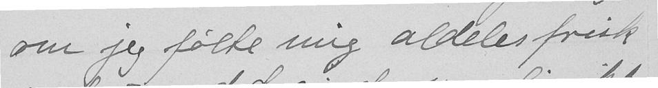om jeg følte mig aldeles frisk
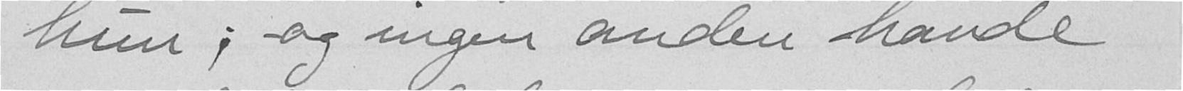hun; og ingen anden havde
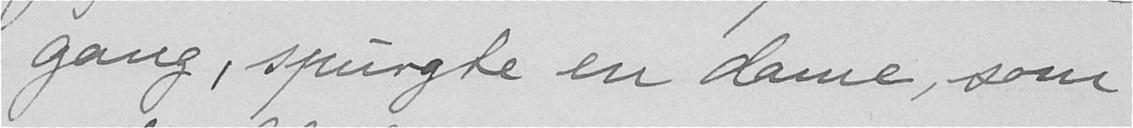gang, spurgte en dame, som
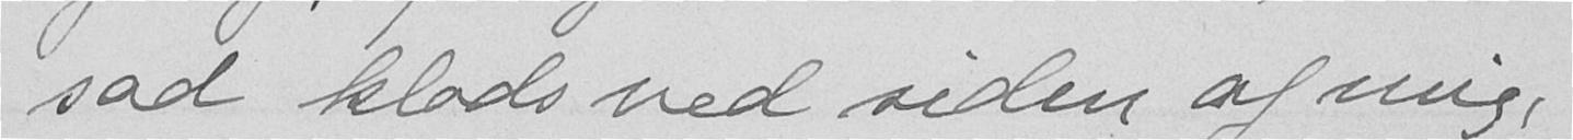sad klods ved siden af mig
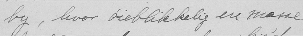by, hvor øieblikkelig en masse
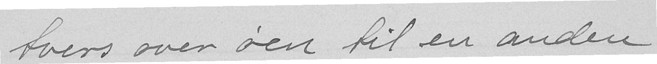tvers over øen til en anden
